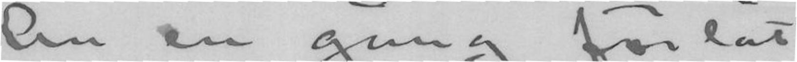Enn en gång forlåt
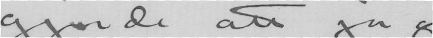gjorde att jag
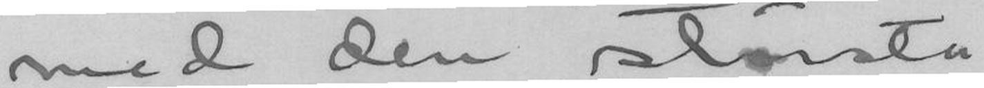med den størsta
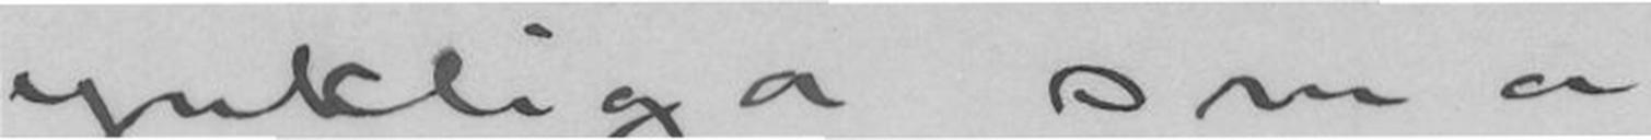ynkeliga små
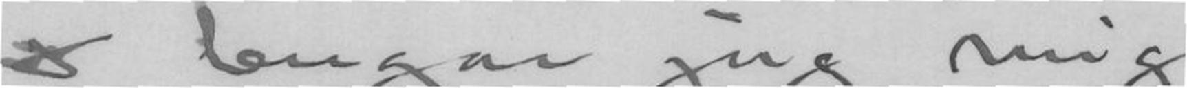& jag mig
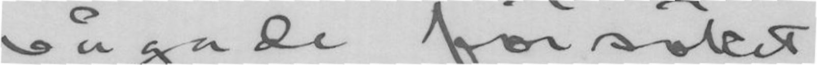vågade försöket
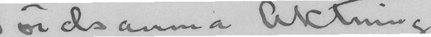Vördsamma Aktning
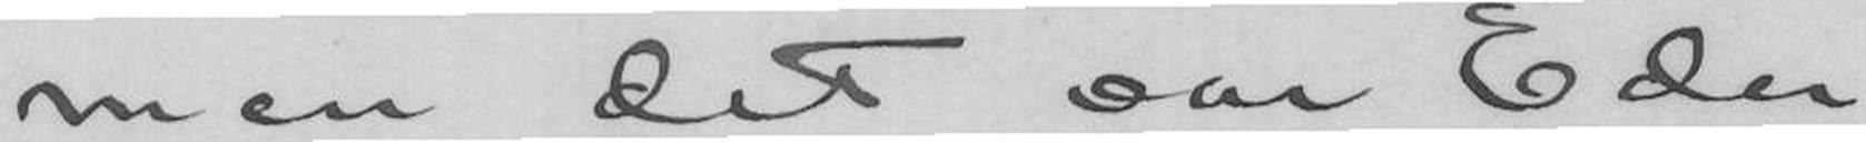men det var Eder
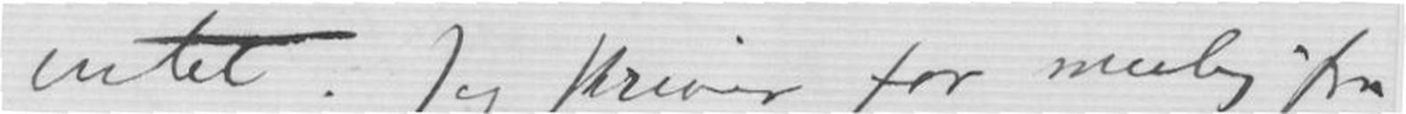intet. Jeg skriver for mulig fra
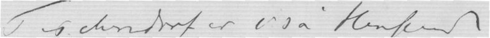af Tischendorf er i så Henseende
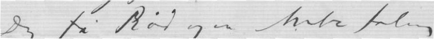Dig få Råd og en Anbefaling
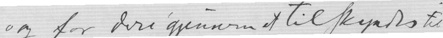og for deri gjennem et tilskapedes til
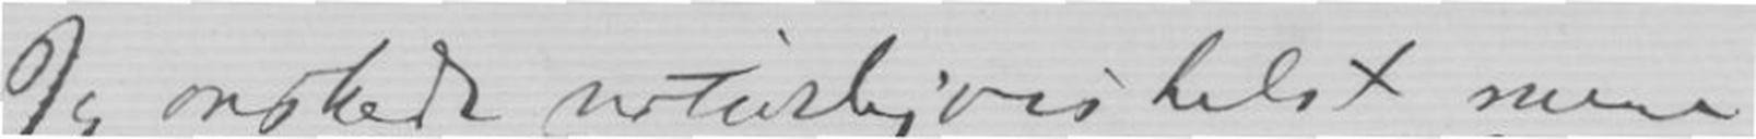Jeg ønkede naturligvis helst mine
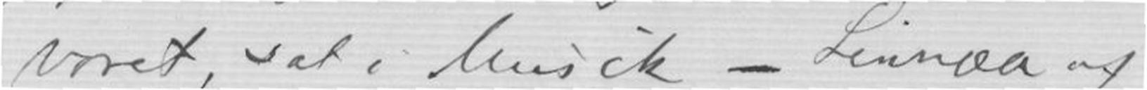været, sat i Musik - Linnæa
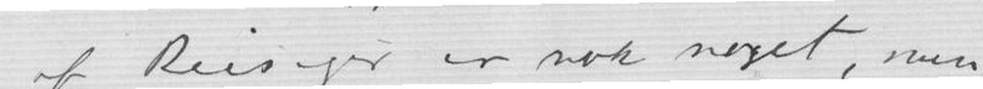af Reiseger er nok noget, men
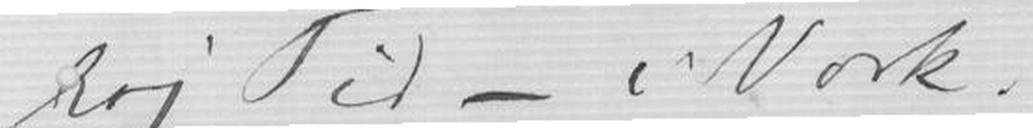høj Tid - i Værk.
